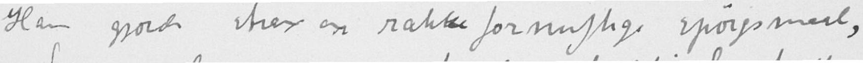Han gjorde strax en række fornuftige spørgsmaal,
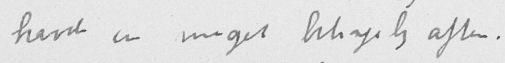havde en meget behagelig aften.
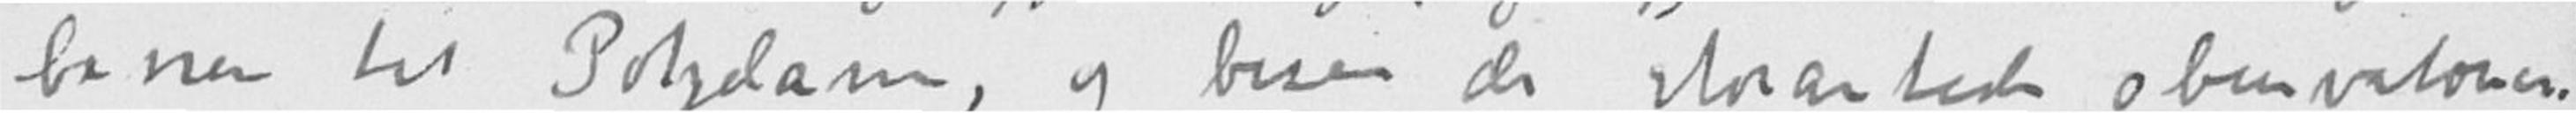banen til Potzdam, og besaa de storartede observatorier.
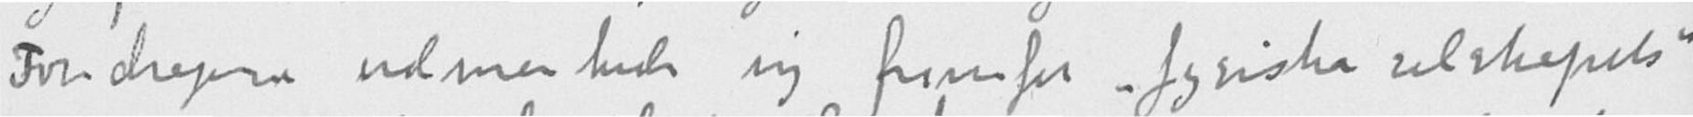Foredragene udmerkede sig fremfor "fysiske selskapets"
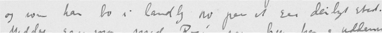og som kan bo i landlig ro paa et saa deiligt sted.
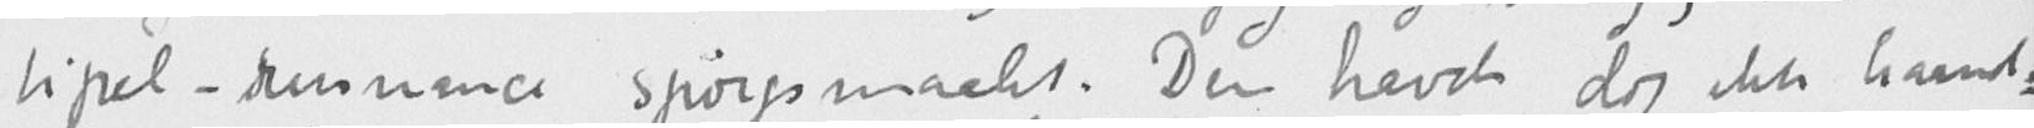tipel-resonance spørgsmaalet. Den havde dog ikke haand-
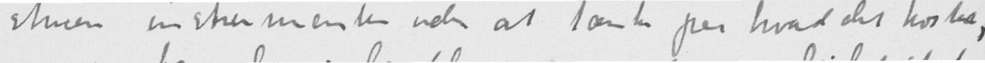struere instrumenter uden at tænke paa hvad det koster,
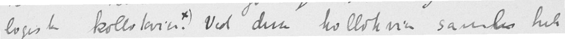logiske kollokvier.*) Ved disse kollokvier samles hele
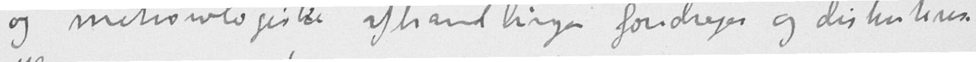og meteorologiske afhandlinger foredrages og diskuteres.
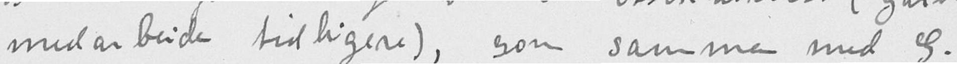medarbeider tidligere), som sammen med G.
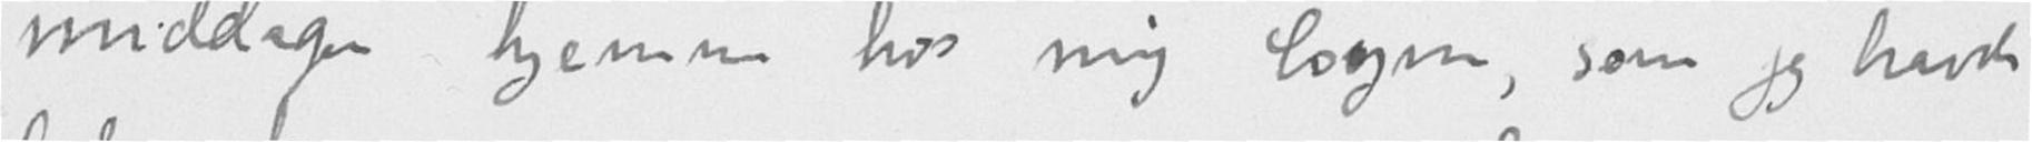middagen hjemme hos mig Coym som jeg havde
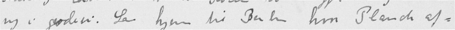sig i goderi. Saa hjem til Berlin hvor Planck af-
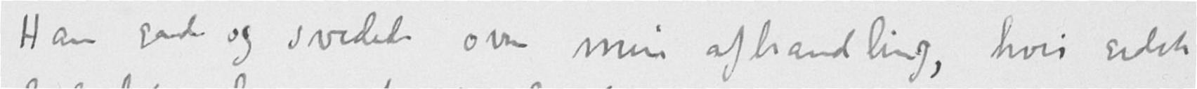Han sad og svedede over min afhandling, hvis sidste
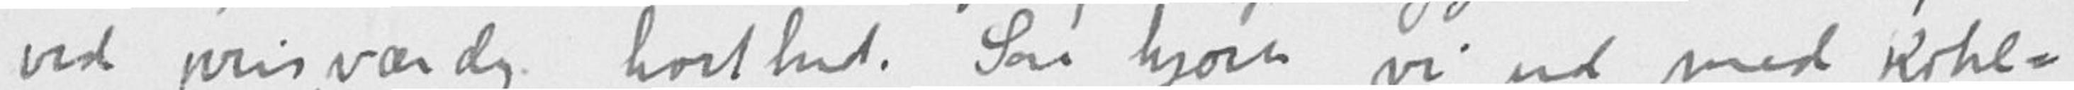ved prisværdig korthed. Saa kjørte vi ud med Kohl-
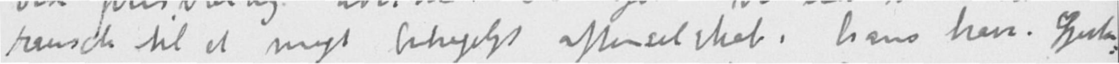rausch til et meget behageligt aftenselskab i hans have. Gjerke
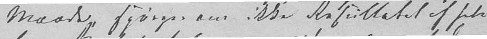Maade, spørges om ikke Resultatet af hele
